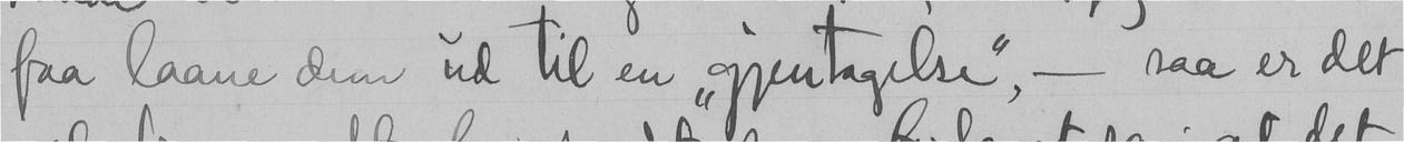faa laane dem ud til en "gjentagelse", - saa er det
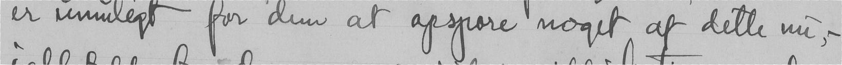er umuligt for dem at opspore noget af dette nu,-
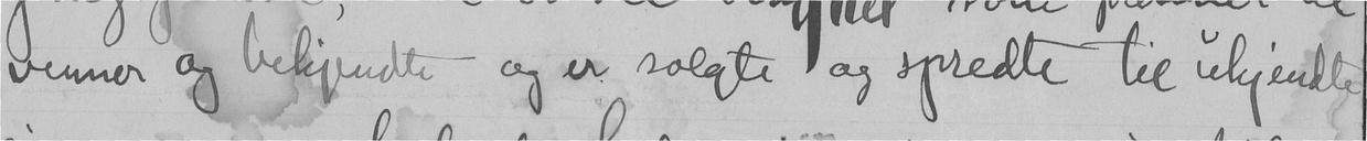venner og bekjendte og er solgte og spredte til ukjendte
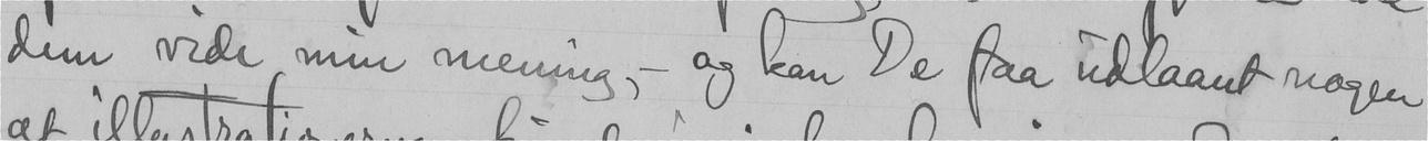dem vide min mening, - og kan De faa udlaant nogen
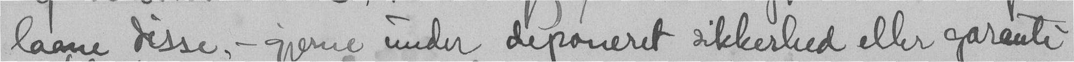laane disse, - gjerne under deponeret sikkerhed eller garanti
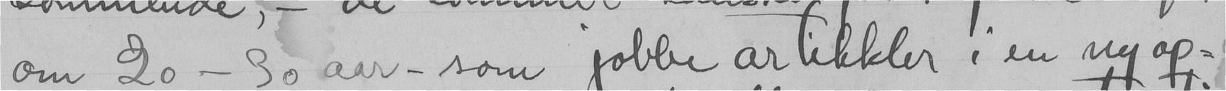om 20-30 aar, - som jobbe artikkler i en ny op-
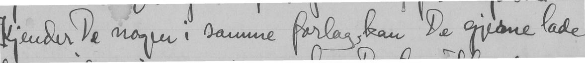Kjender De nogen i samme forlag, kan De gjerne lade
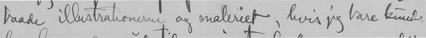baade illustrationerne og maleriet, hvis jeg bare kunde
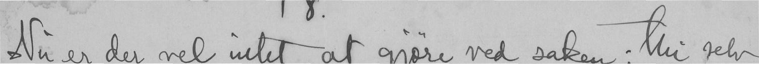Nu er der vel intet at gjøre ved saken; thi selv
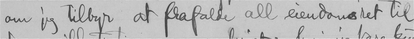om jeg tilbyr at frafalde all eiendomsret til
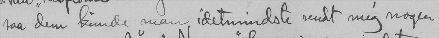saa dem kunde man idetmindste sendt mig nogen
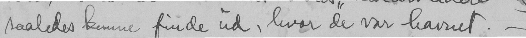saaledes kunne finde ud, hvor de var havnet. -
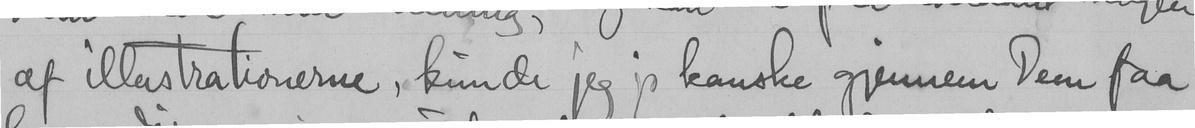af illustrationerne, kunde jeg jo kanske gjennem Dem faa
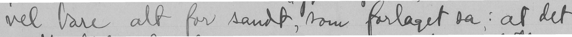vel bare alt for sandt, som forlaget sa : at det
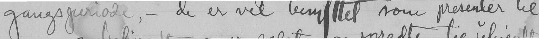gangsperiode, - de er vel benyttet som presenter til
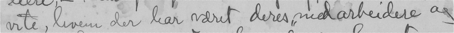vite, hvem der har været deres "medarbeidere" og
